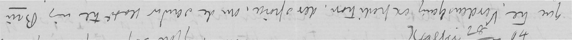gaa til Verdens Gang expedition, der spørge, om de sender bladet til mig Bru
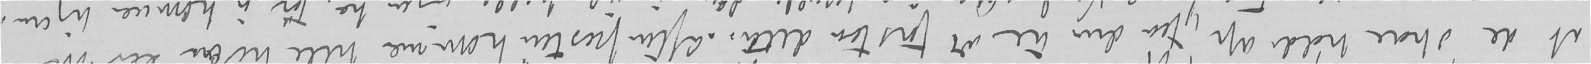at de skal holde op, faa dem til at forstaa dette. . Aftenposten kommer hele tiden Der maa
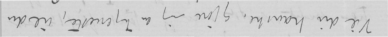Vil du kanske, gjøre mig en tjeneste, vil du
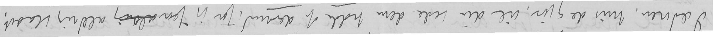Jæderen, hvis de gjør, vil du bede dem holde op dermed, for jeg faa aldrig aldrig bladet,
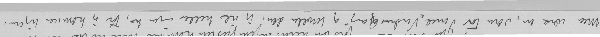maa være en, som tar denne "Verdens Gang" og beholder den, jeg vil heller ingen ha, før jeg kommer hjem.
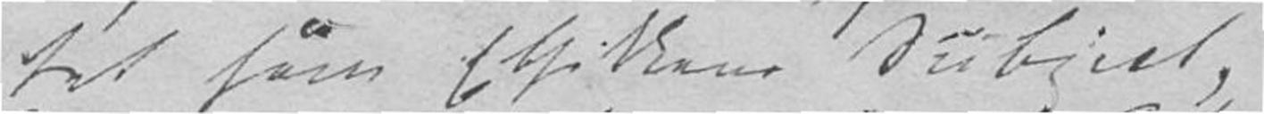tet som Ethikkens Subject,
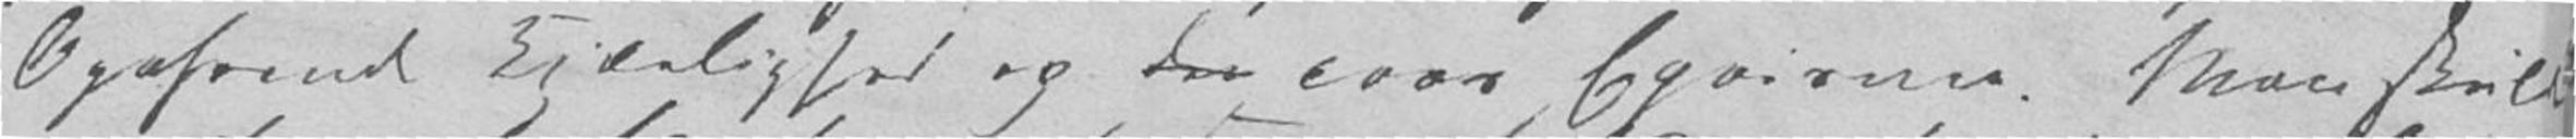Opofrende Kjærlighed og den caar Exiser. Man skulde
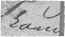kom
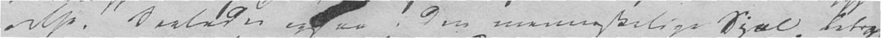relse. Saaledes ogsaa i den menneskelige Sjæl, betrag-
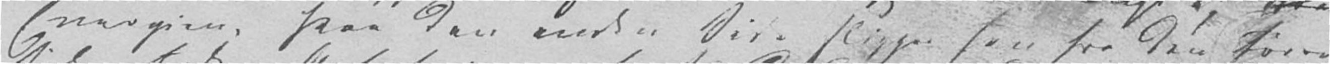Energien. Paa den anden Side slipper han her den før-
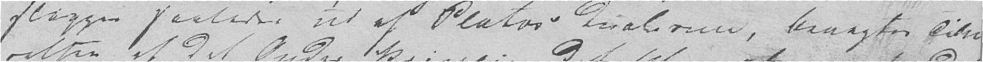slipper saaledes ud af Platos Dilemma, benægter Tilvæ-
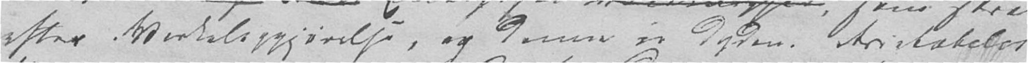efter Virkeliggjørelse, og denne er Dyden. Aristoteles
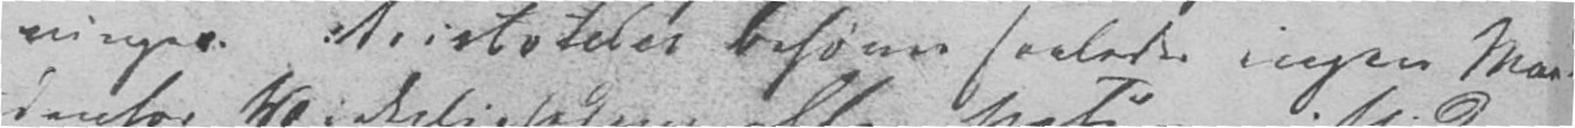ninger. Aristoteles behøver saaledes ingen Maa-
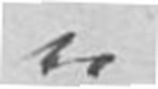er
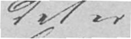det er-
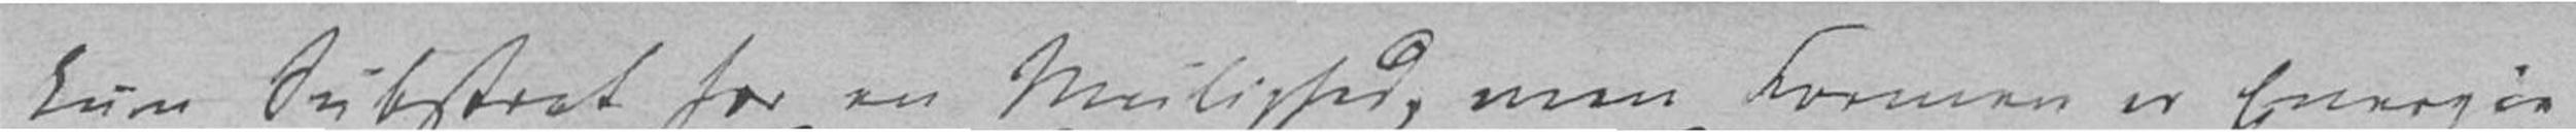kun Substrat for en Mulighed, men Formen er Energie
